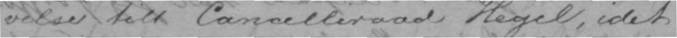velse till Cancelliraad Hegel, idet
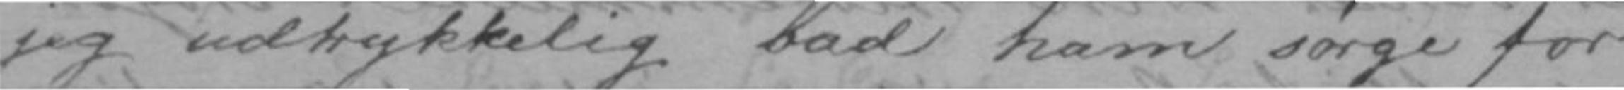jeg udtrykkelig bad ham sørge for
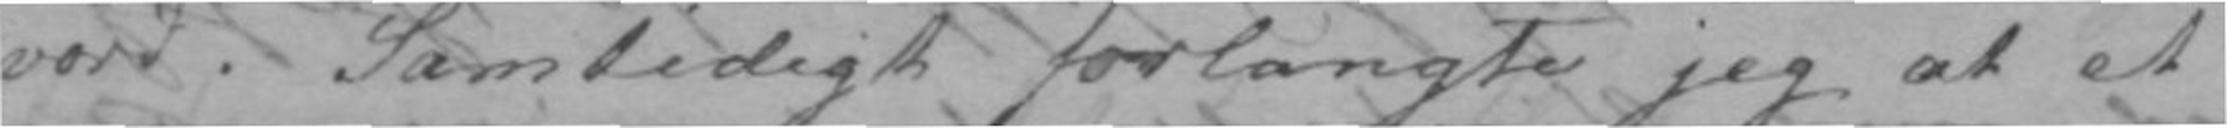værd. Samtidigt forlangte jeg at et
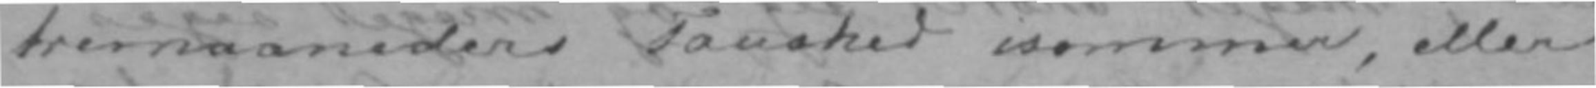tremaaneders Taushed isommer, eller
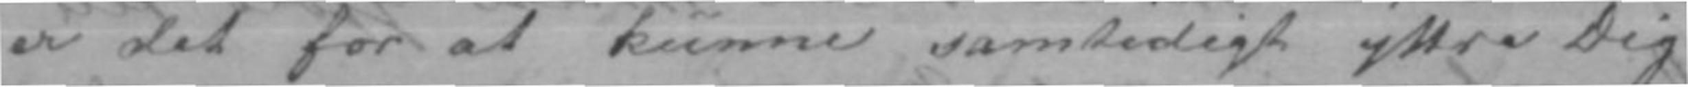er det for at kunne samtidigt yttre Dig
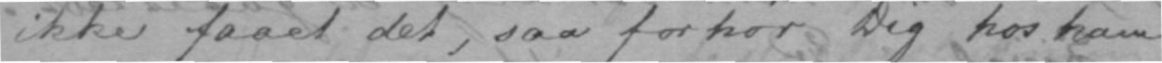ikke faaet det, saa forhør Dig hos ham
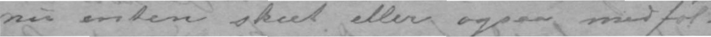nu enten skeet eller ogsaa medføl-
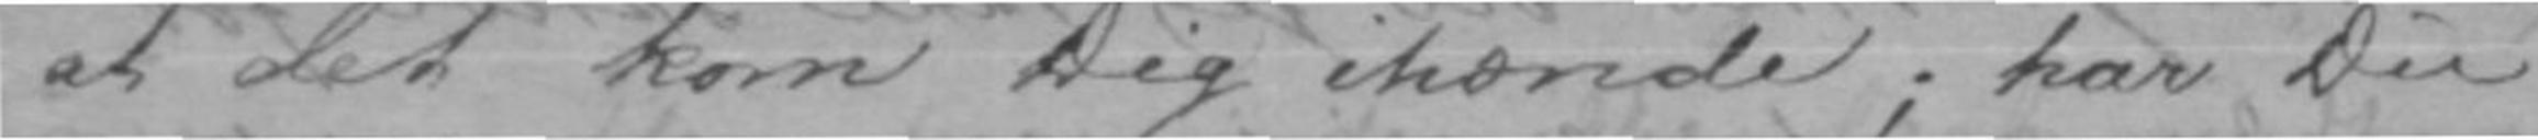at det kom Dig ihænde; har Du
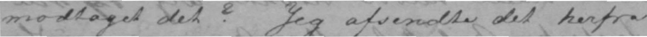modtaget det? Jeg afsendte det herfra
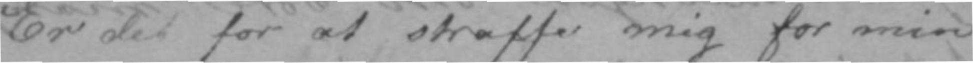Er det for at straffe mig for min
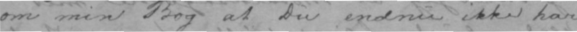om min Bog at Du endnu ikke har
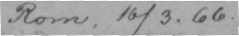Rom, 16/3. 66.
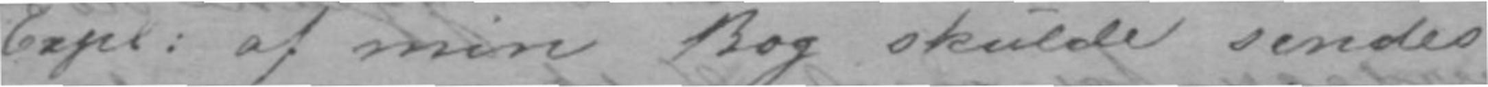Expl: af min Bog skulde sendes
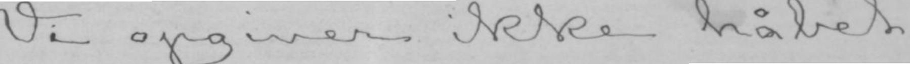Vi opgiver ikke håbet
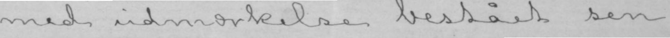med udmærkelse bestået sin
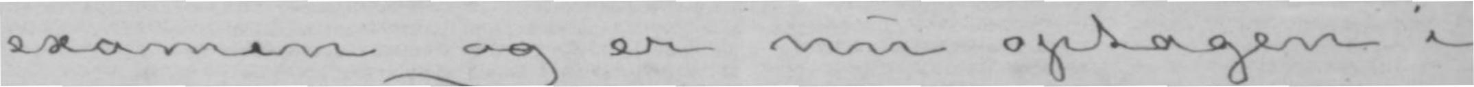examen og er nu optagen i
